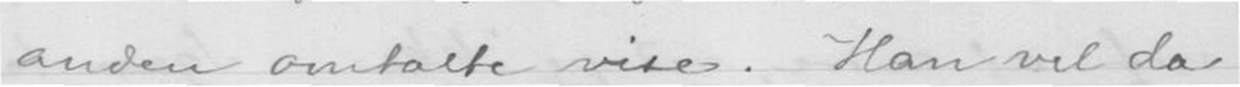anden omtalte vise. Han vil da
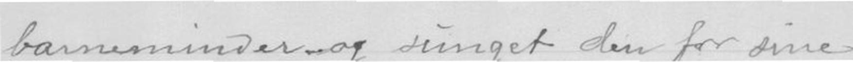barneminder sunget den for sine
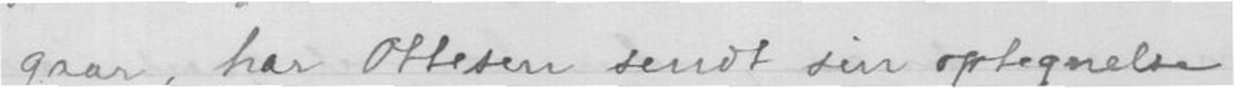gaar, har Ottesen sendt optegnelsen
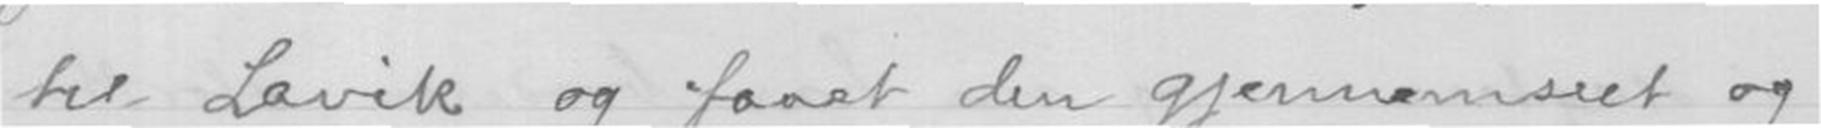til Lavik og faaet den gjennemseet og
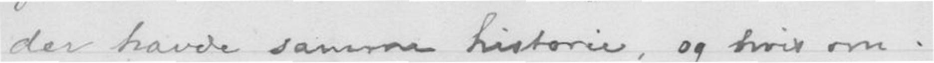der havde samme historie, og hvis om
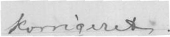korrigeret. -
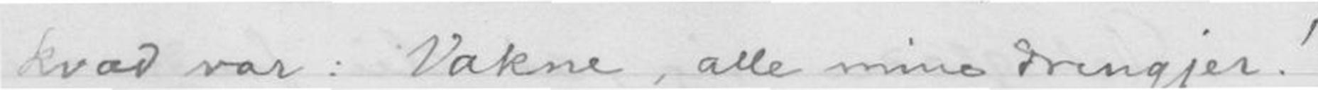kvad var: Vakne, alle mine drengjer!
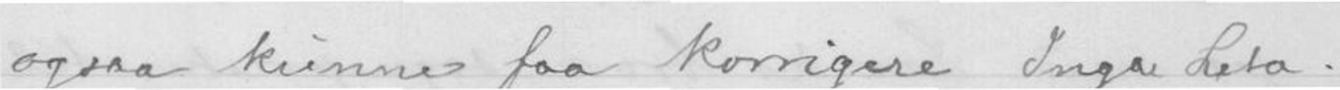ogsaa kunne faa korrigere Inga Lita-
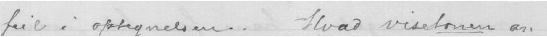feil i optegnelsen. Hvad visetonen an-
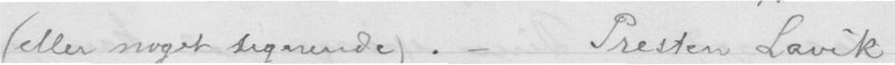(eller noget lignende). - Presten Lavik
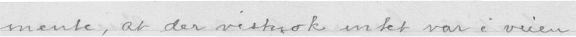mente, at der vistnok intet var i veien
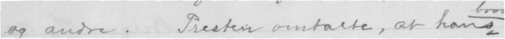og andre. Presten omtalte, at hans
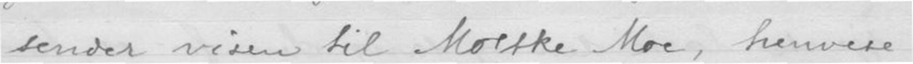sender visen til Moltke Moe, henvise
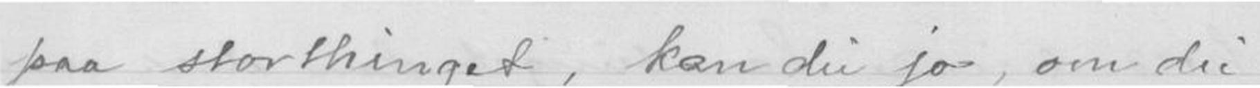paa storthinget, kan du jo, om du
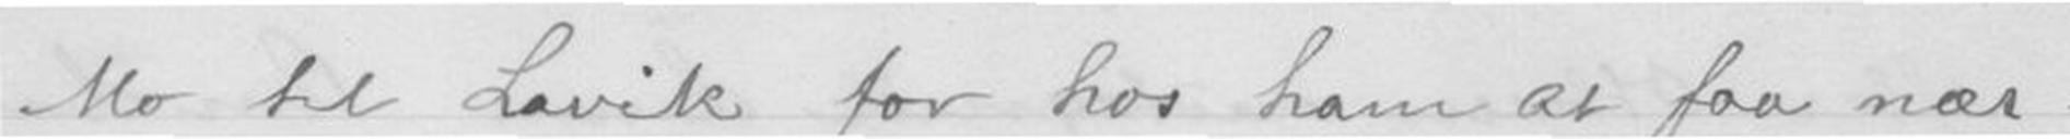Mo til Lavik for hos ham at faa nær-
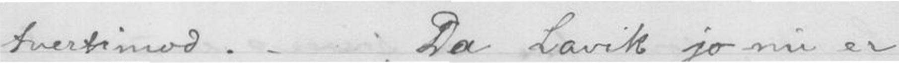tvertimod. - Da Lavik jo nu er
